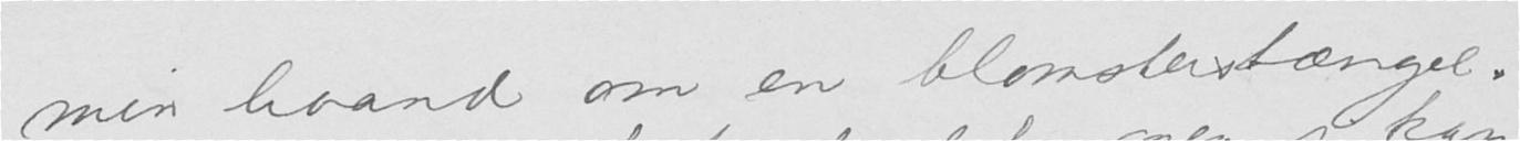min haand om en blomsterstængel.
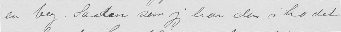en bog. Saadan som jeg har den i hodet
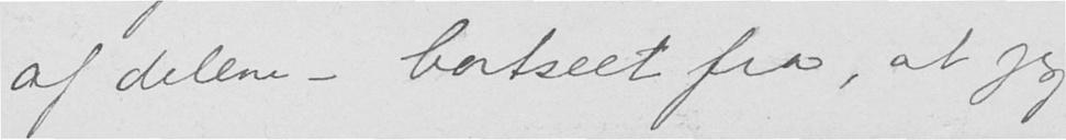af delene - bortseet fra, at jeg
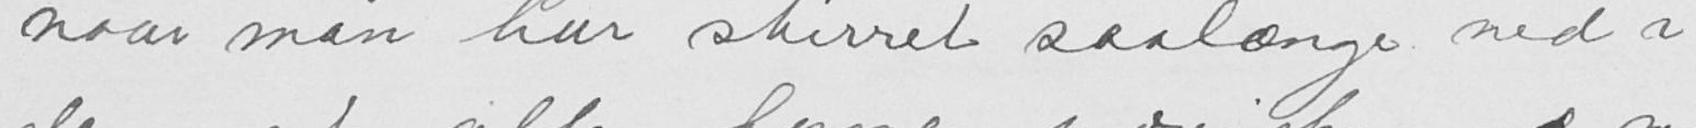naar man har stirret saalænge ned i
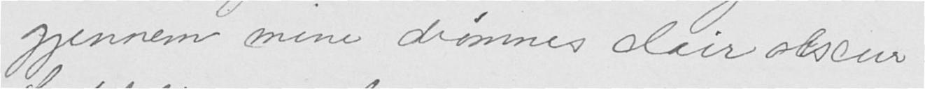gjennem mine drømmers clair obscur.
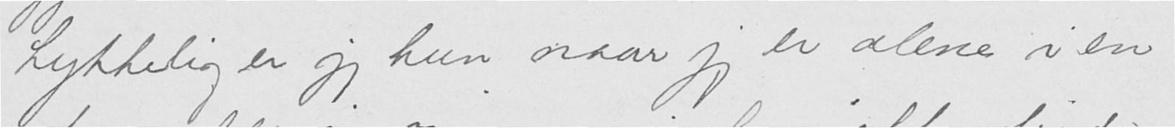Lykkelig er jeg kun naar jeg er alene i en
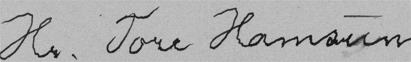Hr. Tore Hamsun
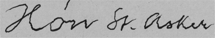Høn St. Asker
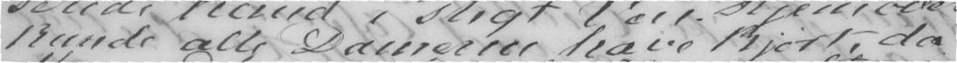kunde alle Damerne have kjørt, da
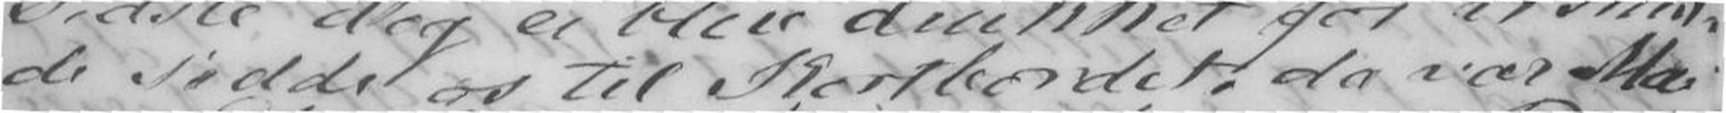de sidde os til Kortbordet, da var Ma-
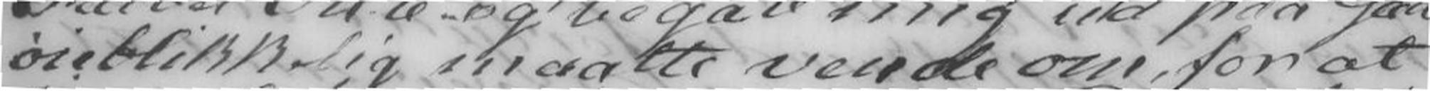øieblikkelig maatte vende om for at
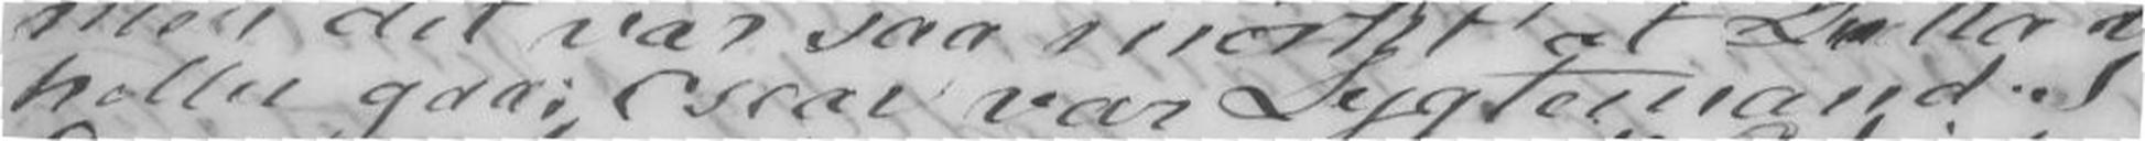heller gaa; Oscar var Lygtemand. I
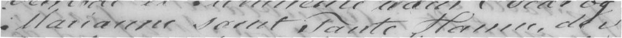Marianne samt Tante Hanne, der
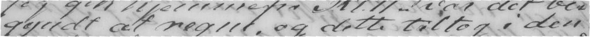gyndt at regne, og dette tiltog i den
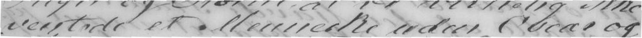ventede et Menneske uden Oscar og
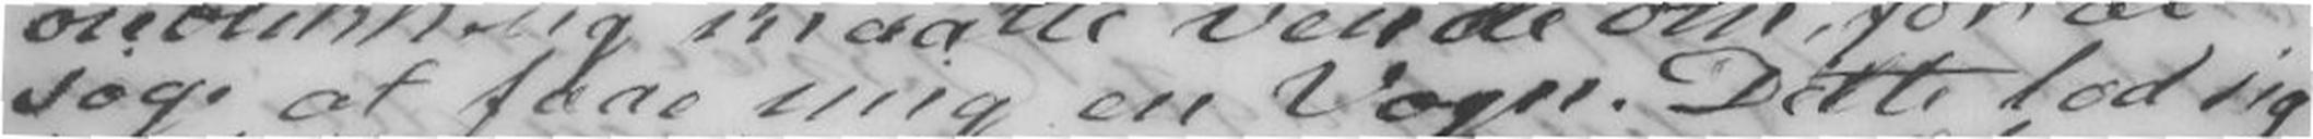søge at faae mig en Vogn. dette lod sig
